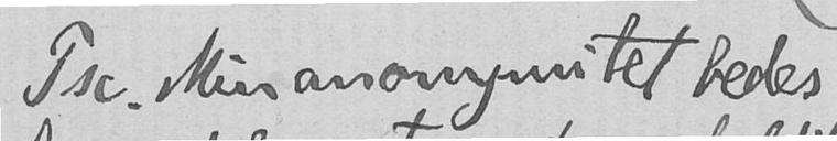P sc. Min anonymitet bedes
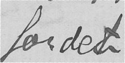for det
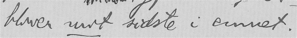bliver mit sidste i emnet.
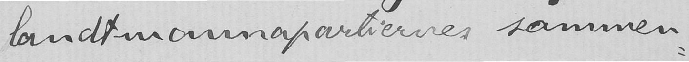landtmannapartiernes sammen-
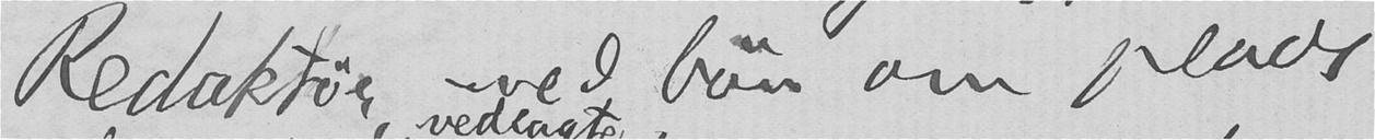Redaktør, ved bøn om plads
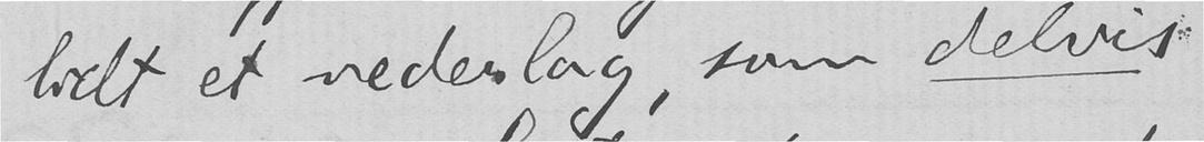lidt et nederlag, som delvis
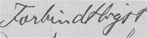Forbindtligst
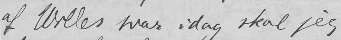af Willes svar idag skal jeg
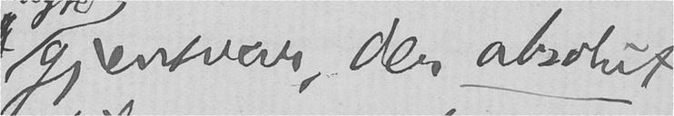gjensvar, der absolut
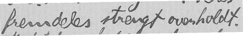fremdeles strengt overholdt.
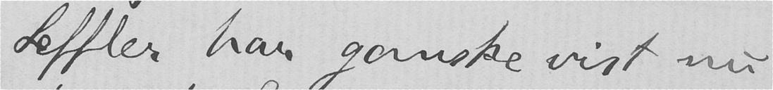Leffler har ganske vist nu
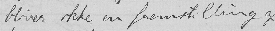bliver ikke en fremstilling af
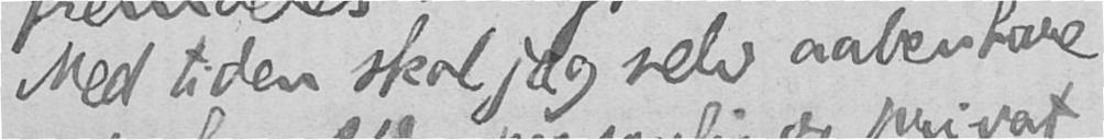Med tiden skal jeg selv aabenbare
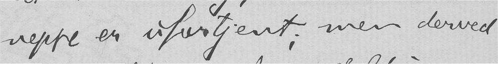neppe er ufortjent; men derved
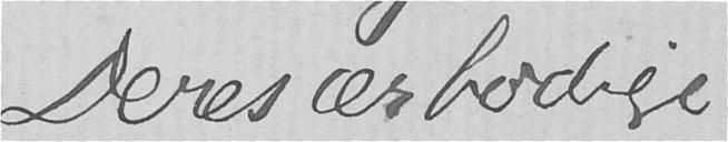Deres ærbødige
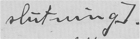slutning].
